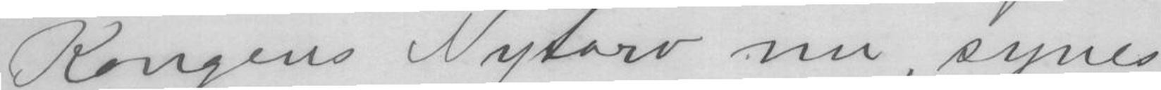Kongens Nytorv nu, synes
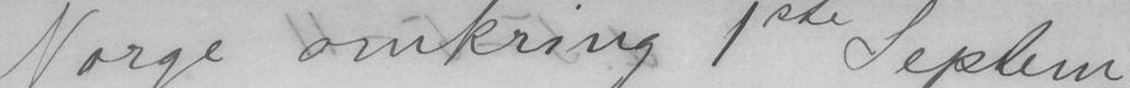Norge omkring 1ste Septem-
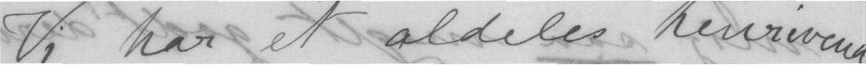Vi har et aldeles henrivende
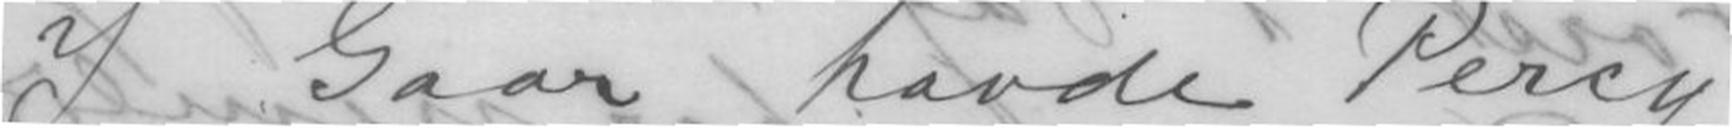I Gaar havde Percy
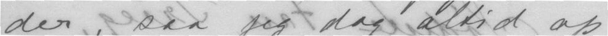der, saa jeg dog altid op
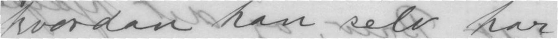hvordan han selv har
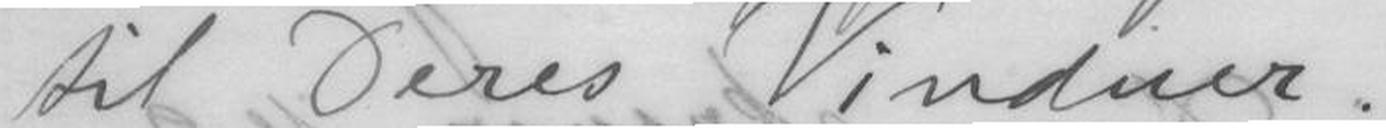til Deres Vinduer.-
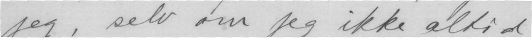jeg, selv om jeg ikke altid
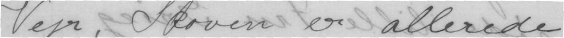Vejr, Skoven er allerede
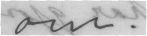om.
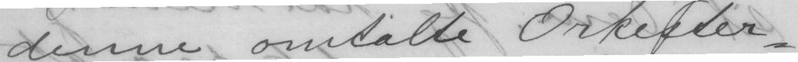denne omtalte Orkester-
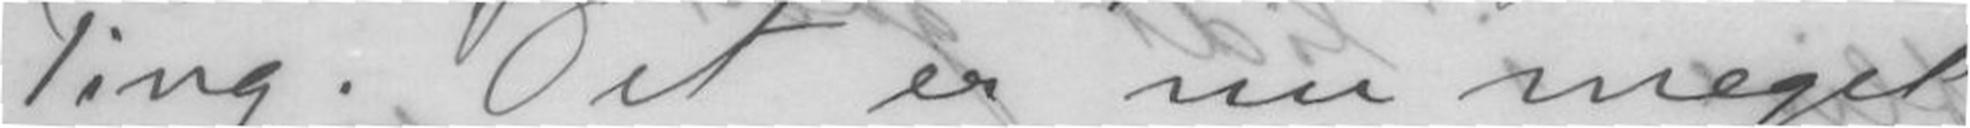Ting. Det er nu meget
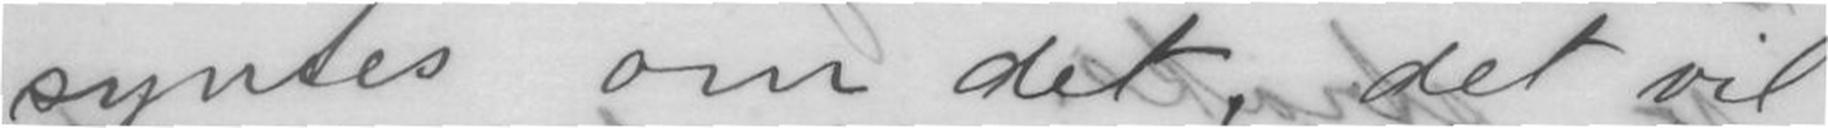syntes om det, det vil
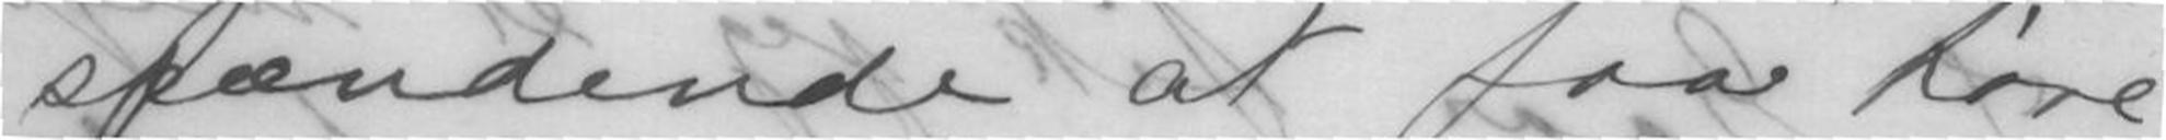spændende at faa høre
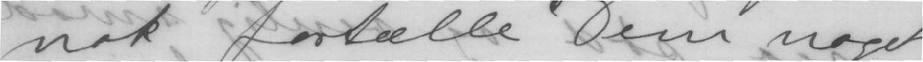nok fortælle Dem noget
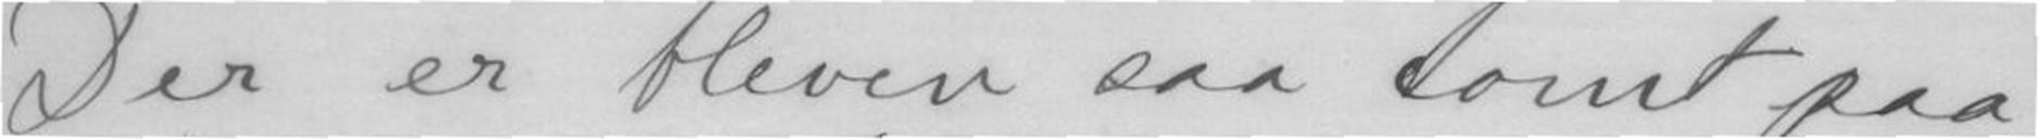Der er bleven saa tomt paa
